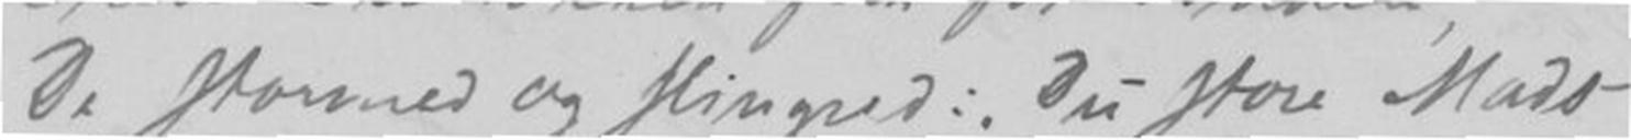De stormed og slingred:, Du store Mads -
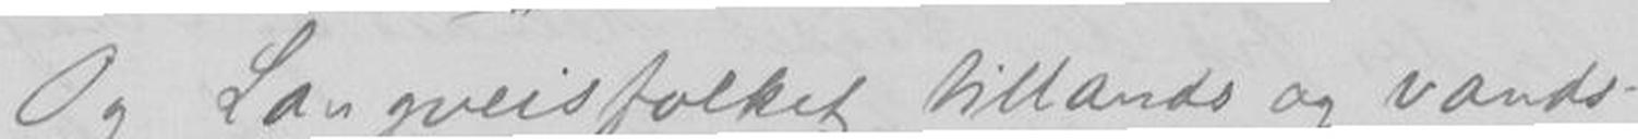Og Langveisfolket tillands og vands.
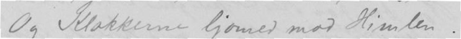Og Klokkerne ljomed mod Himlen!
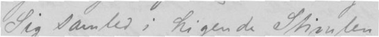Sig samled i higende Stimler -
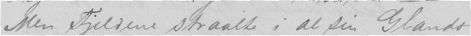Men Fjeldene straalte i al sin Glands
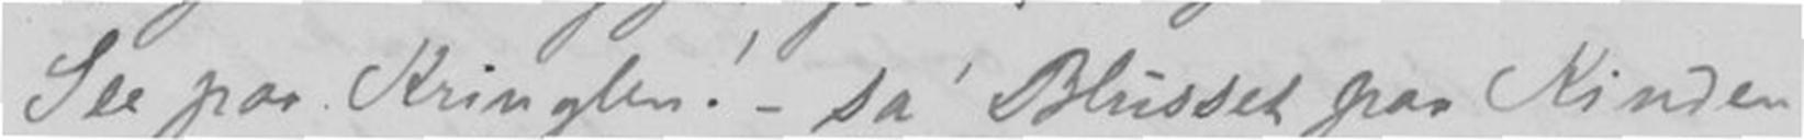See paa Kringlen! - sa' Blusset paa Kinden
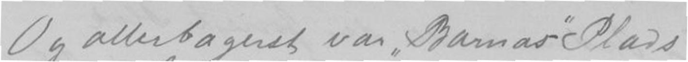Og allerbagerst var Barnas Plads
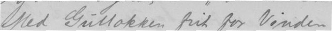Med Gullokker frit for Vinden,
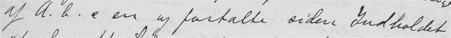af A.b.e en og fortalte siden Indholdet
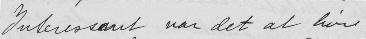Interessant var det at høre
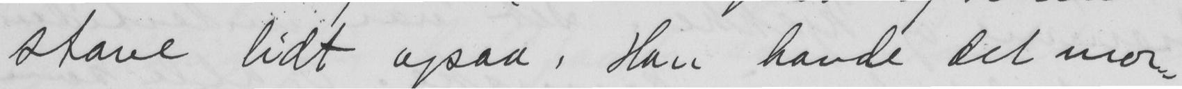stave lidt ogsaa. Han havde det mor-
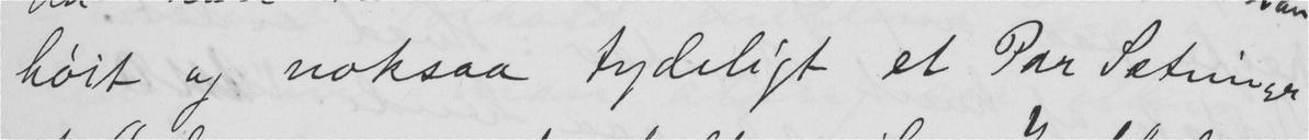høit og noksaa tydeligt et Par Sætninger.
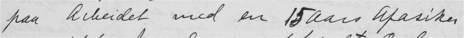paa Arbeidet med en 15 Aars Afasiker
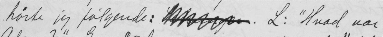hørte jeg tølgende:  L. "Hvad var
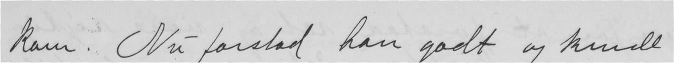kom. Nu forstod han godt og kunde
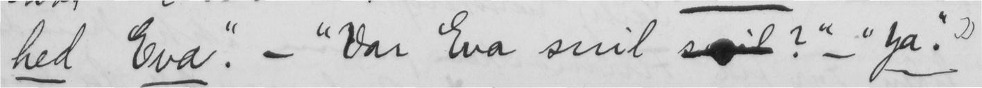hed Eva". - "Var Eva snil ?" - "Ja".
